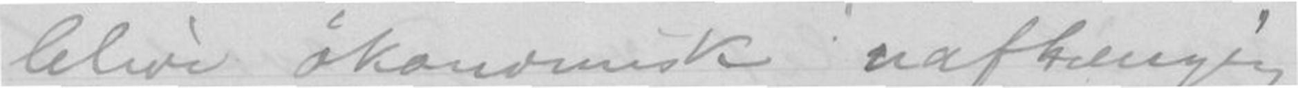blive økonomisk uafhengig
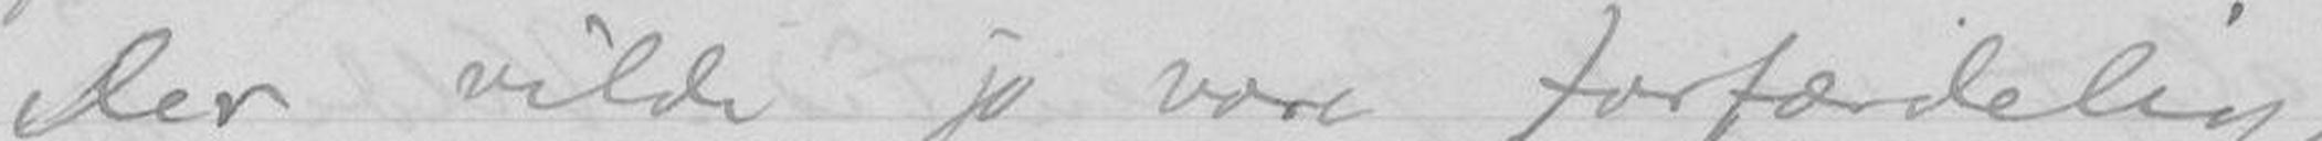Der vilde jo være forfærdelig
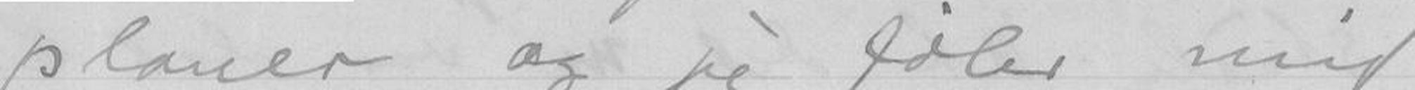planer og jeg føler mig
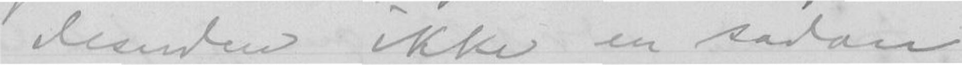desuden ikke en sådan
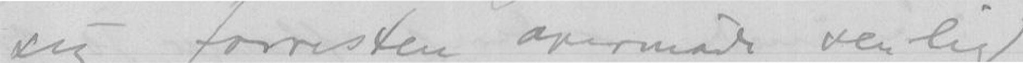sig forresten overmåde venlig
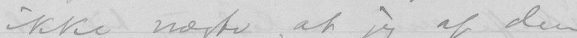ikke nægte, at jeg af den
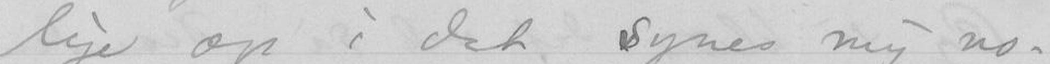lige op i det synes mig no-
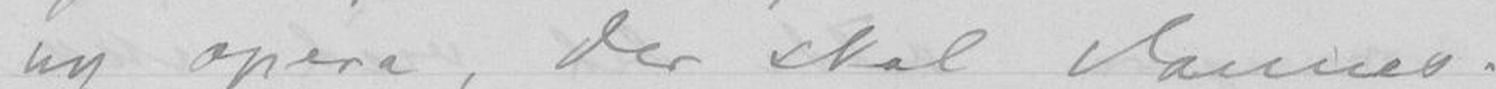ny opera, der skal dannes
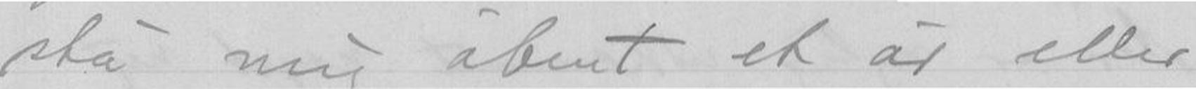stå mig åbent et år eller
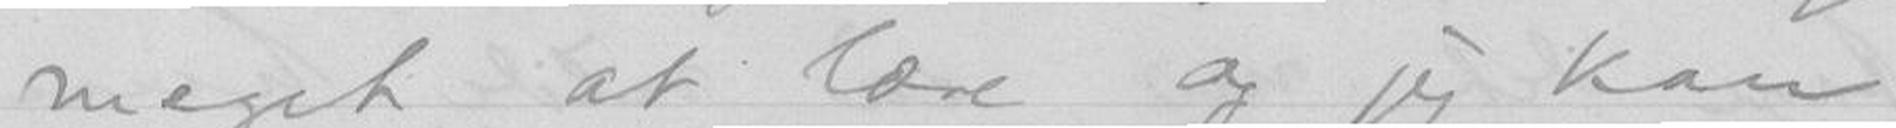meget at lære og jeg kan
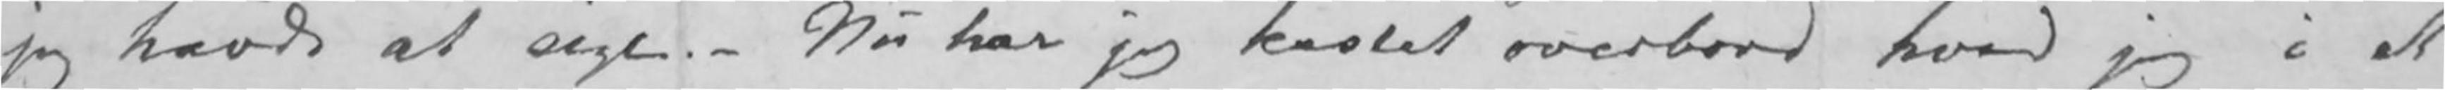jeg havde at sige.- Nu har jeg kastet overbord hvad jeg i et
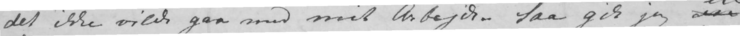det ikke vilde gaa med mit Arbejde. Saa gik jeg en
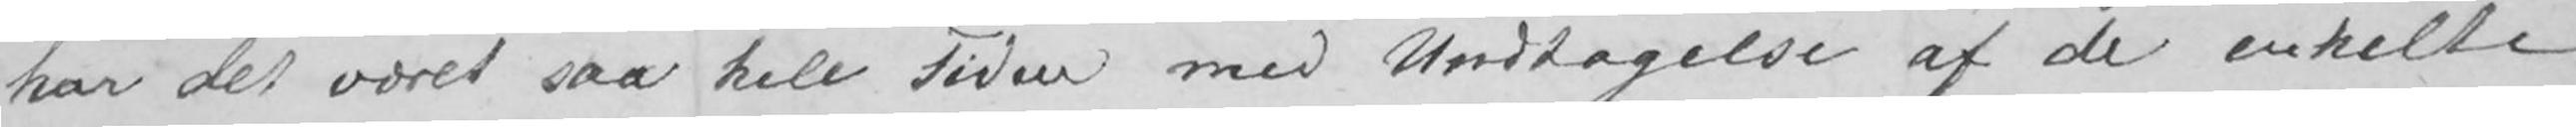har det været saa hele Tiden med Undtagelse af de enkelte
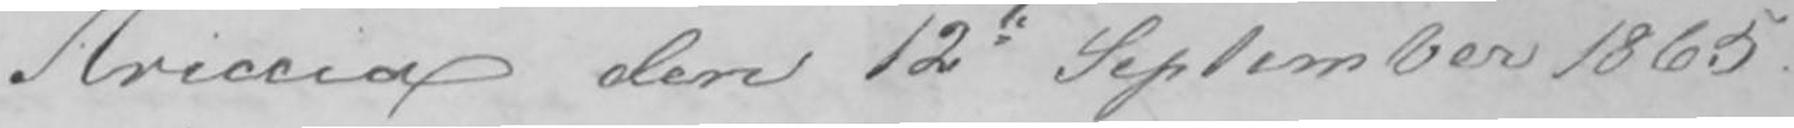Ariccia den 12te September 1865.
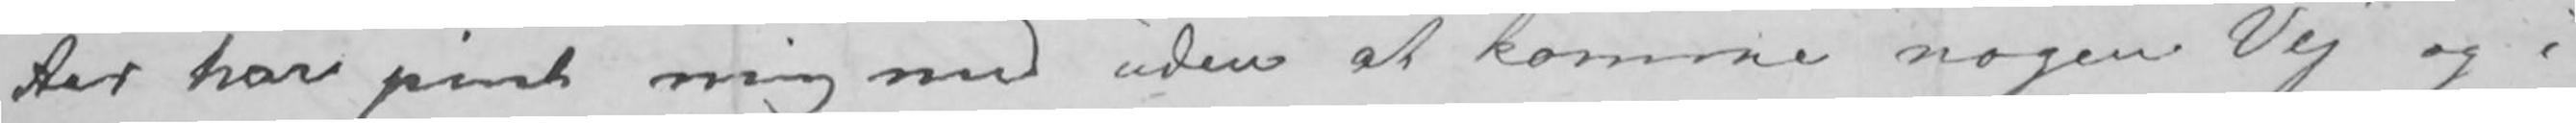Aar har pint mig med uden at komme nogen Vej og i
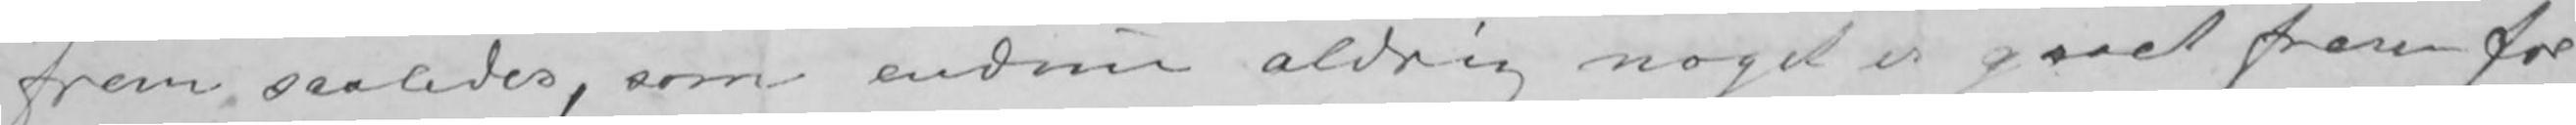frem saaledes, som endnu aldrig noget er gaaet frem for
